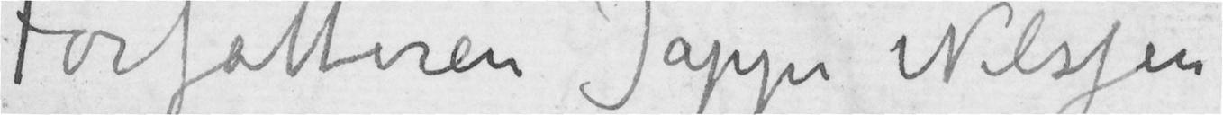Forfatteren Jappe Nilssen
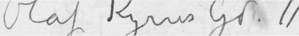Olaf Kyrres Gd. 11
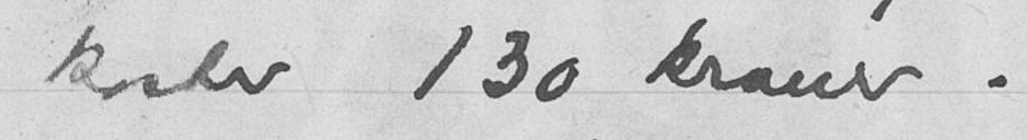koster 130 kroner.
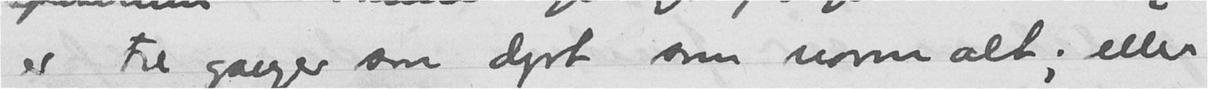er tre ganger saa dyrt som normalt; eller
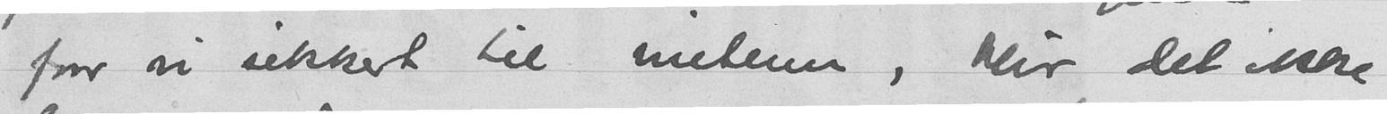faar vi sikkert til imellem, blir det ikke
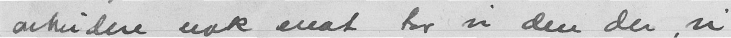arbeidere nok snart for vi den der, vi
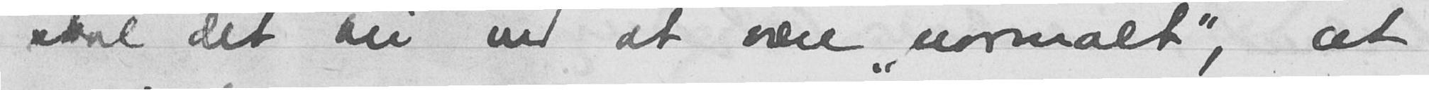skal det bli ved at være "normalt", at
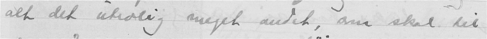alt det utrolig meget andet, som skal til
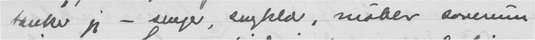tænker jeg - senger, sengklær, møbler soverum
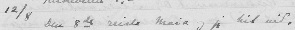12/8 Den 8de reiste Maia og jeg hit ind,
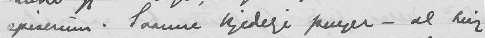spiserum. Saanne kjedelige penger - al ting
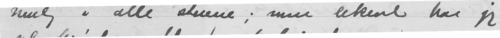mulig i alle stuene; men likevel har jeg
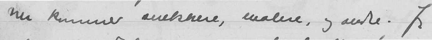nu kommer snekkere, malere, og andre. Jeg
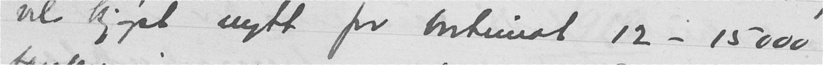vel kjøpt nytt for bortimot 12-15000
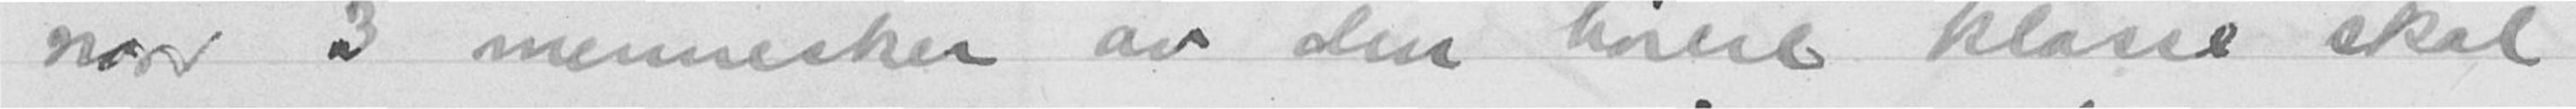naar 3 mennesker av den høiere klasse skal
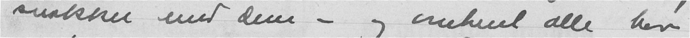snakker med dem - og omtrent alle har
